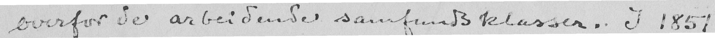overfor de arbeidende samfundsklasser.. I 1851
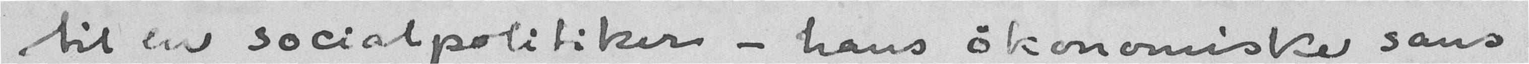til en socialpolitiker - hans økonomiske sans
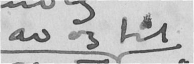av og til
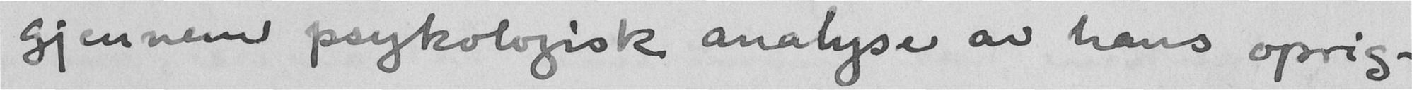gjennem psykologisk analyse av hans oprig-
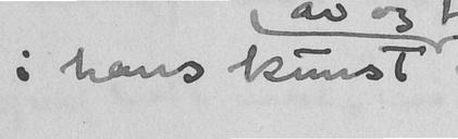i hans kunst
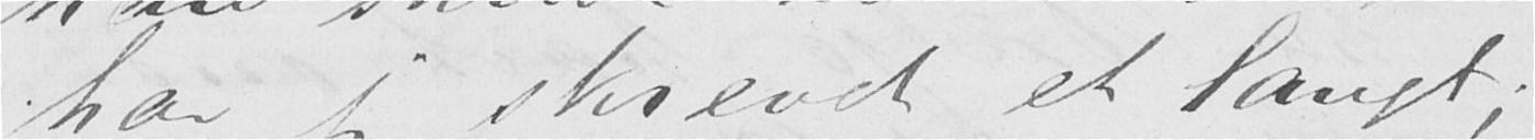har jeg skrevet et langt;
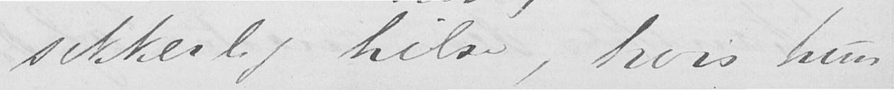sikkerlig hilse, hvis hun
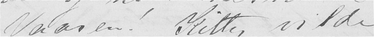Vaaren! Kitty vilde
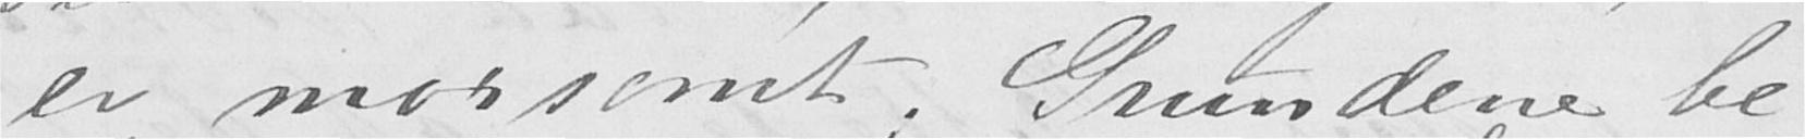er morsomt. Grundene be-
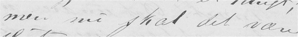men nu skal det være
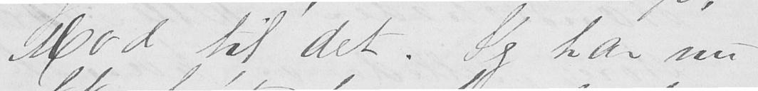Mod til det. Jeg har nu
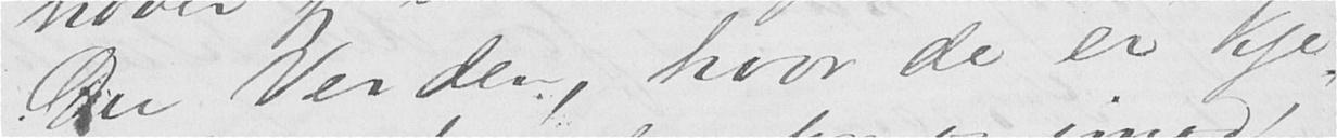Du Verden, hvor de er kje-
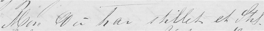Mod Du har stillet et Stil-
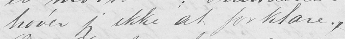høver jeg ikke at forklare.
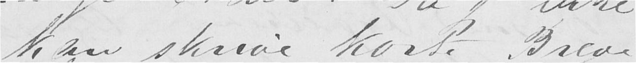kan skrive korte Breve
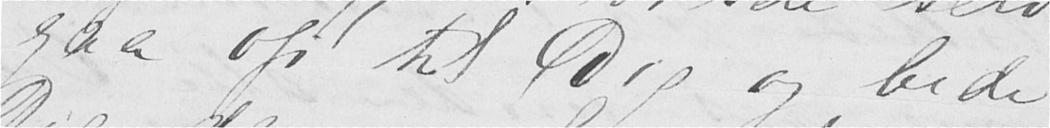gaa op til Dig og bede
